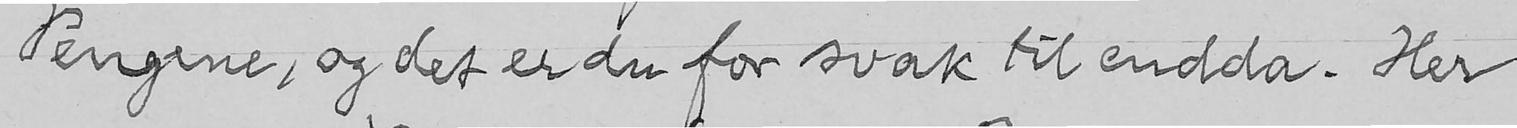Pengene, og det er du for svak til endda. Her
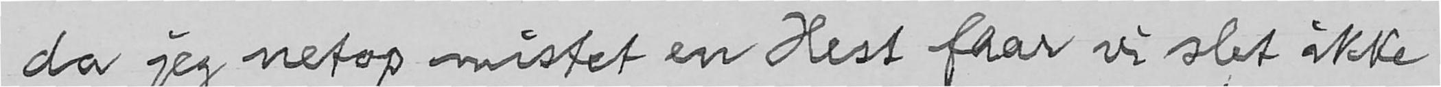da jeg netop mistet en Hest faar vi slet ikke
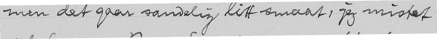men det gaar sandelig litt smaat, jeg mistet
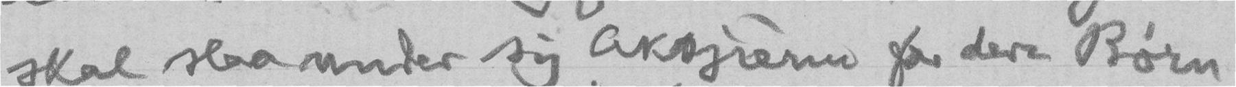skal slaa under sig Aksjierne for dere Børn
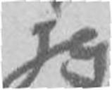jeg
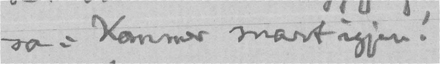sa: Kommer snart igjen!
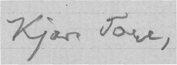Kjære Tore,
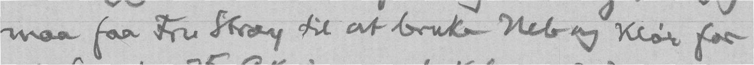maa faa Fru Stray til at bruke Neb og Klør for
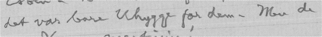det var bare Uhygge for dem. Men de
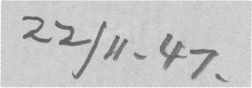22/11. 47.
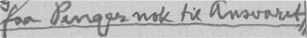faa Penger nok til Ansvaret"
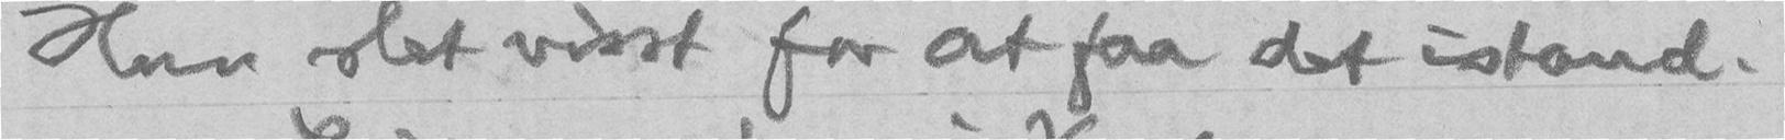Hun slet visst for at faa det istand.
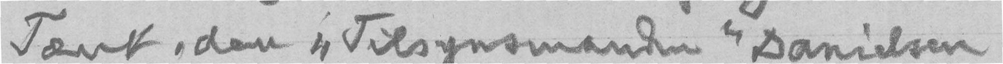Tænk, den "Tilsynsmanden" Danielsen
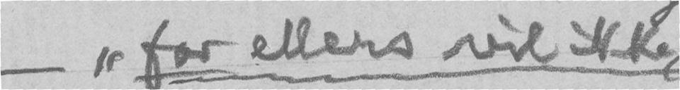- "for ellers vil ikke
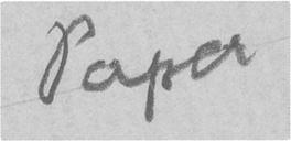Papa
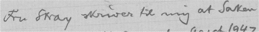Fru Stray skriver til mig at Saken
